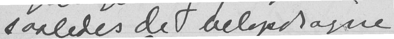saaledes de velopdragne
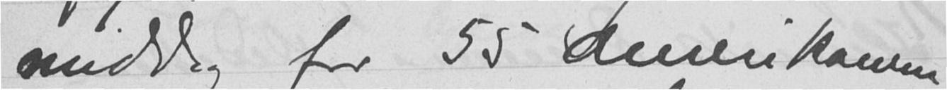middag for 55 amerikanere
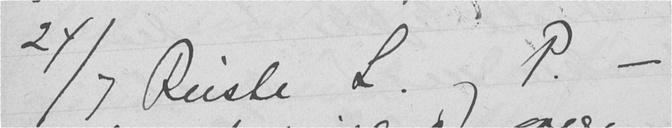24/7 Reiste L. og P. -
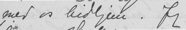med os hidhjem. Jeg
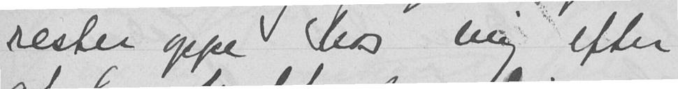rester oppe hos mig efter
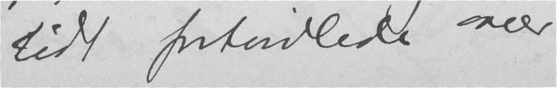lidt fortvivlede over
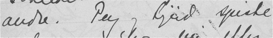andre. Pey og Sigrid spiste
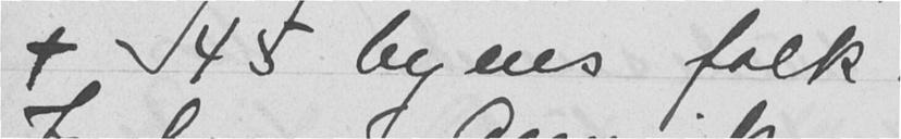+ 45 byens folk.
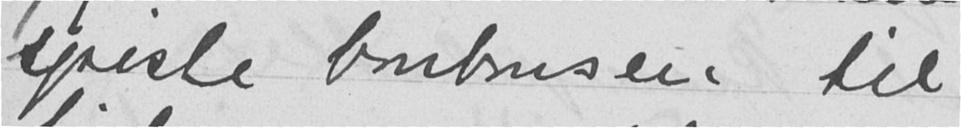spiste bonbonser til
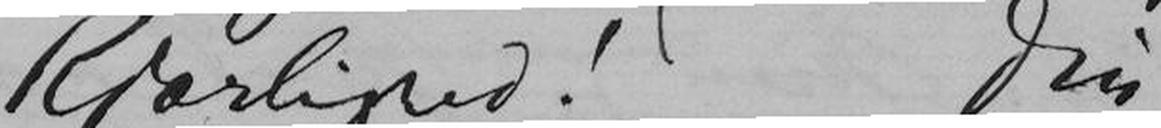Kjærlighed! Din
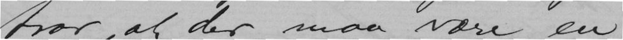tror, at der maa være en
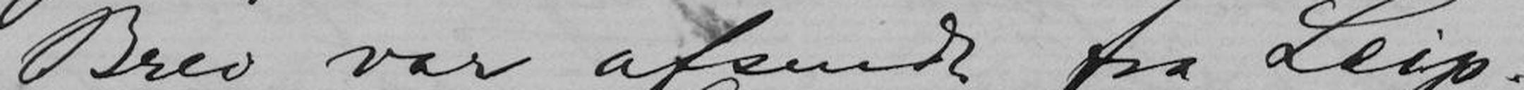Brev var afsendt fra Leip-
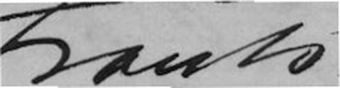Frants.
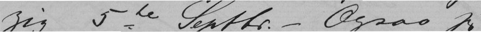zig 5te Septbr. - Ogsaa jeg
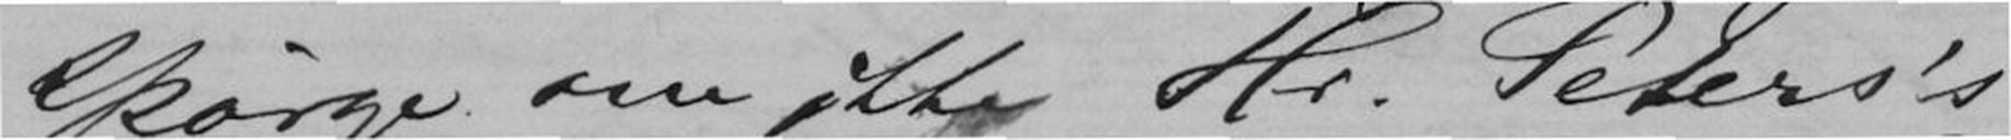spørge om ikke Hr. Peters's
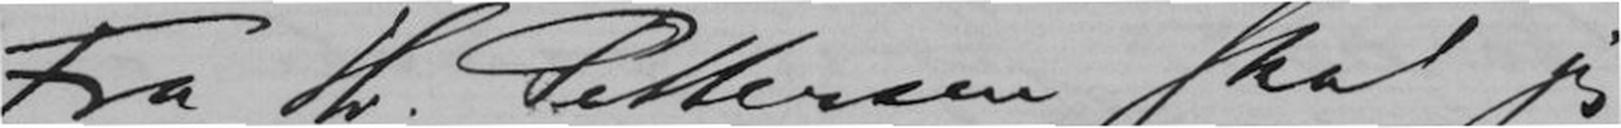Fra Hr. Pettersen skal jeg
